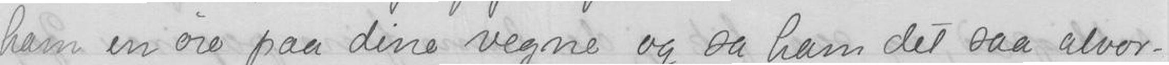ham en øre paa dine vegne og sa ham det saa alvor-
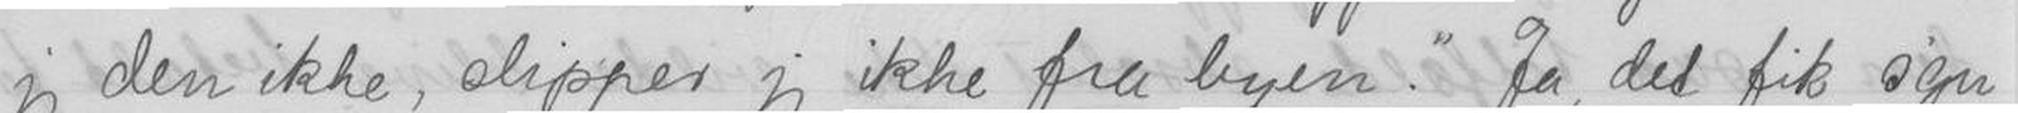jeg den ikke, slipper jeg ikke fra byen. Ja, det fik sgu
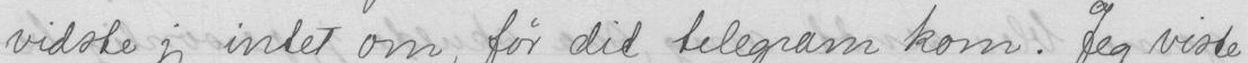vidste jeg intet om, før dit telegram kom. Jeg viste
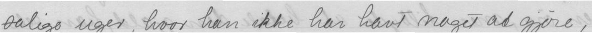salige uger, hvor han ikke har havt noget at gjøre,
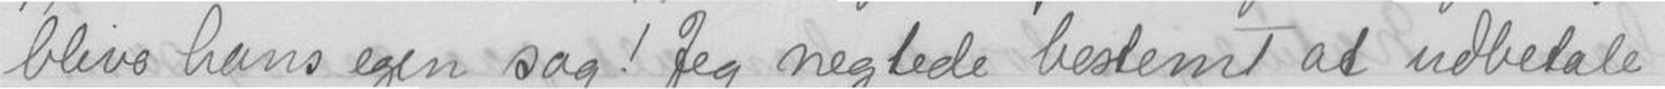blive hans egen sag! Jeg negtede bestemt at udbetale
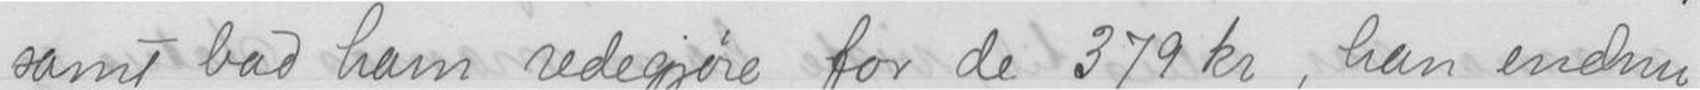samt bad ham redegjøre for de 379 kr, han endnu
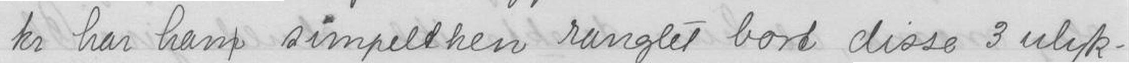kr har ham simpelthen ranglet bort disse 3 ulyk-
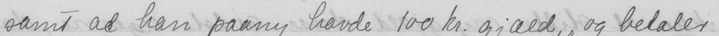samt at han paany havde 100 kr. gjæld, og betaler
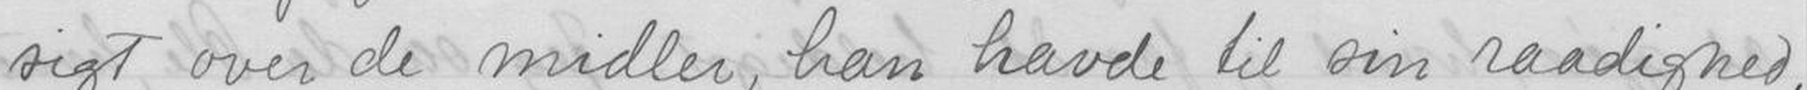sigt over de midler, han havde til sin raadighed,
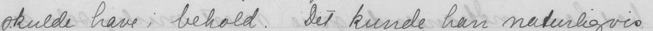skulde have i behold. Det kunde han naturligvis
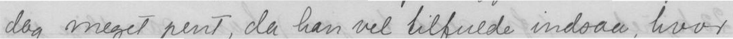dog meget pent, da han vel tilfulde indsaa, hvor
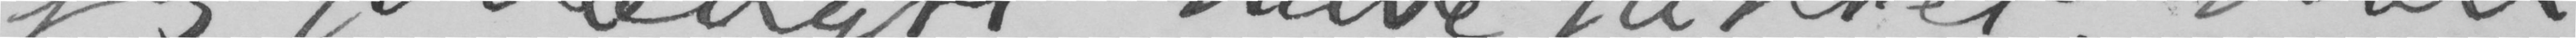jeg forlængst have takket. Men
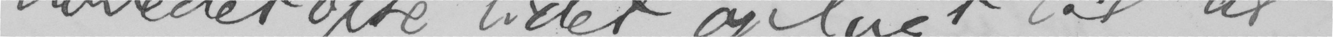hovedet ofte lidet oplagt til at
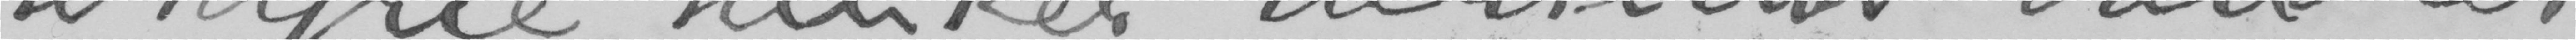toldfrie tanker derimod vandrer
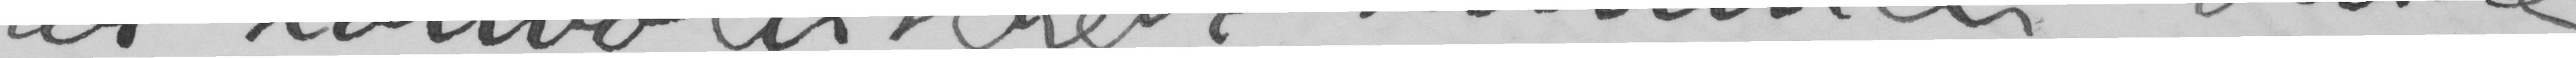ar, konvoluterede sammen, burde
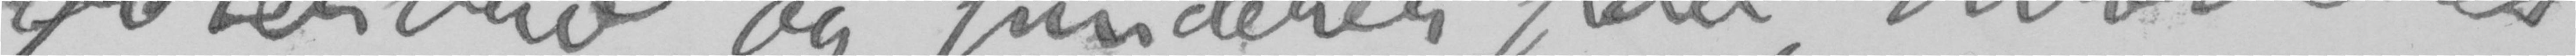Østerbro og funderer paa, hvorledes
